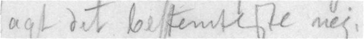sagt det bestemteste nej.
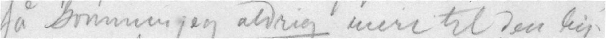så kommer jeg aldrig mere til den by.
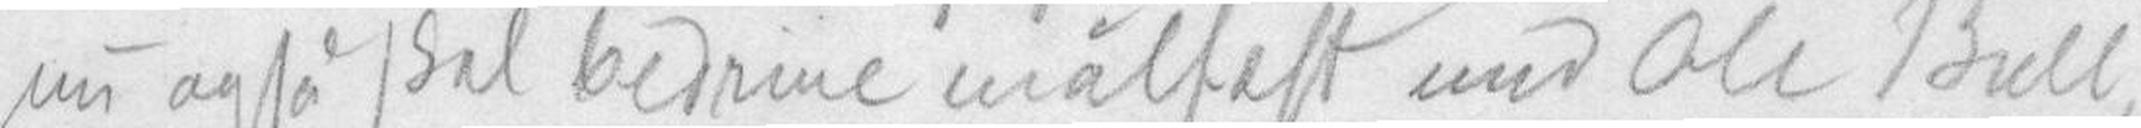nu også skal bedrive målfest med Ole Bull,
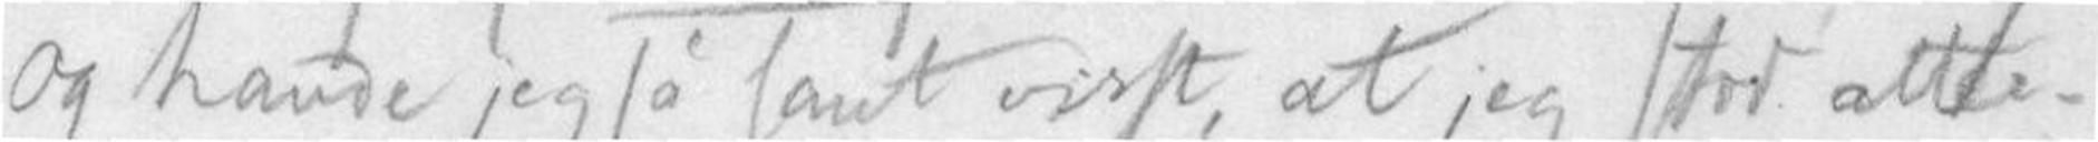Og havde jeg så sant visst, at jeg stod alter-
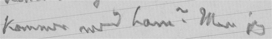kommer med ham? Men jeg
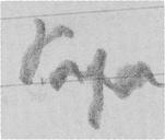Papa
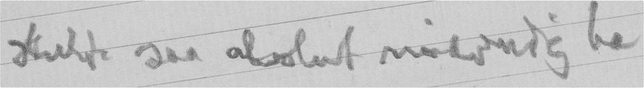skulde saa absolut nødvendig ha
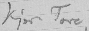Kjære Tore,
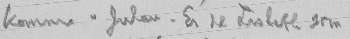komme i Julen. Er det Lisbeth som
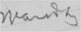Mandag
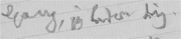Gang, jeg beder dig.
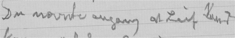Du nævnte engang at Leif kunde
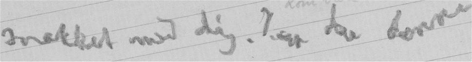snakket med dig. Kom bare denne
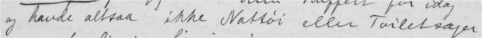og havde altsaa ikke Nattøi eller Toiletsager
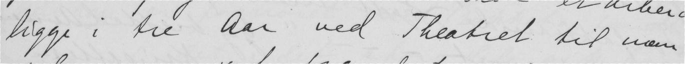ligge i tie Aar ved Theatret til man
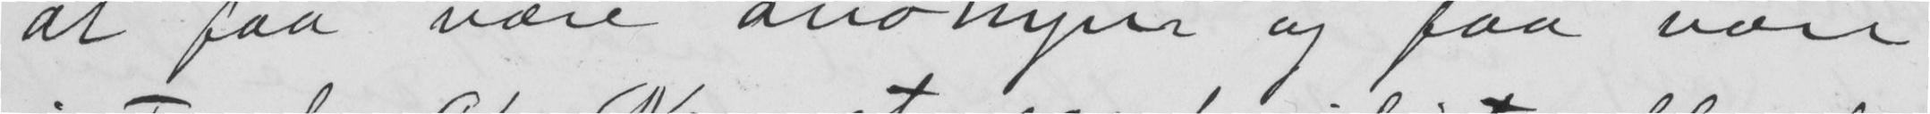at faa være anonym og faa være
